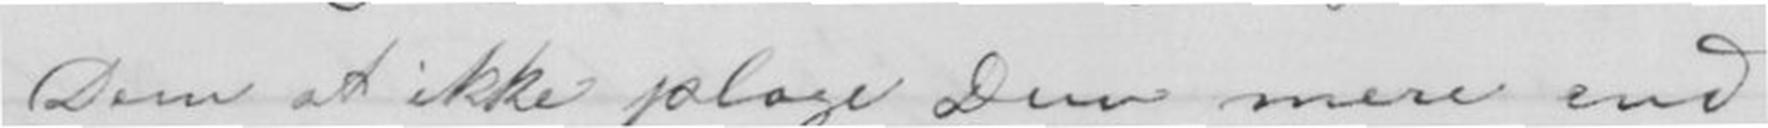Dem at ikke plage Dem mere end
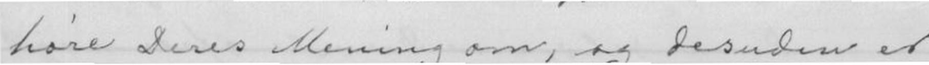høre Deres Mening om, og desuden er
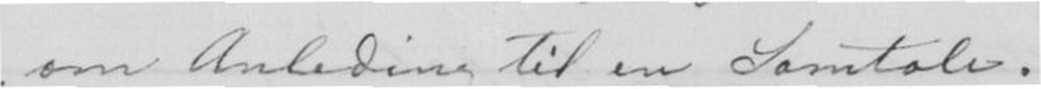om Anledning til en Samtale.
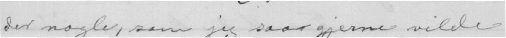der nogle, som jeg saa gjerne vilde
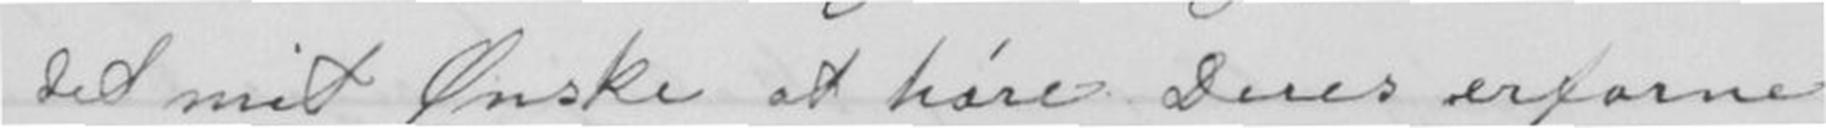det mit Ønske at høre Deres erfarne
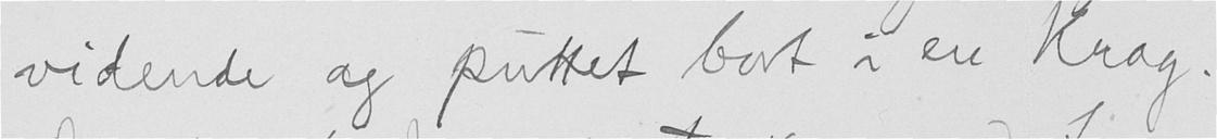vidende og puttet bort i en Krog.
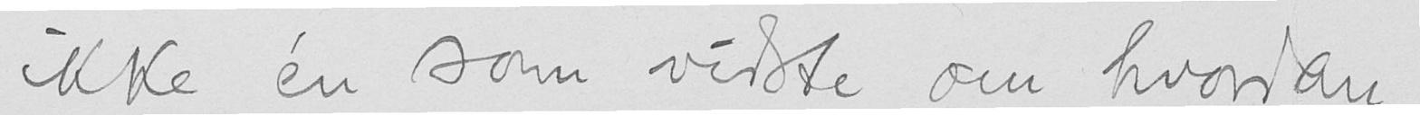ikke én som vidste om hvordan
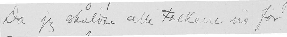Da jeg skældte alle Folkene ud for
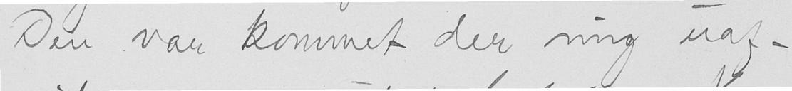Den var kommet der mig uaf-
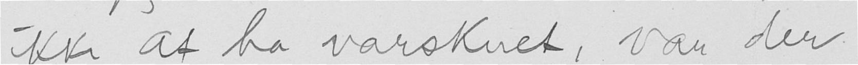ikke at ha varskuet, var der
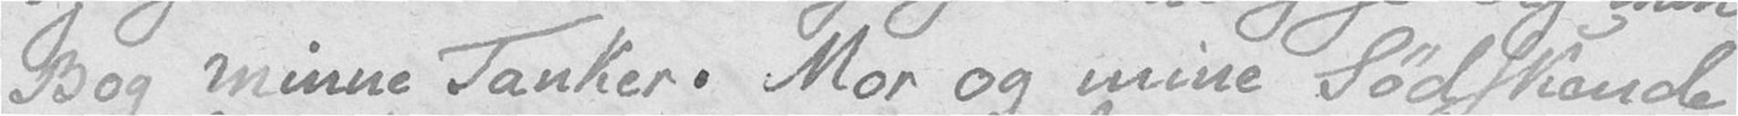Bog minne Tanker. Mor og mine Sødskende
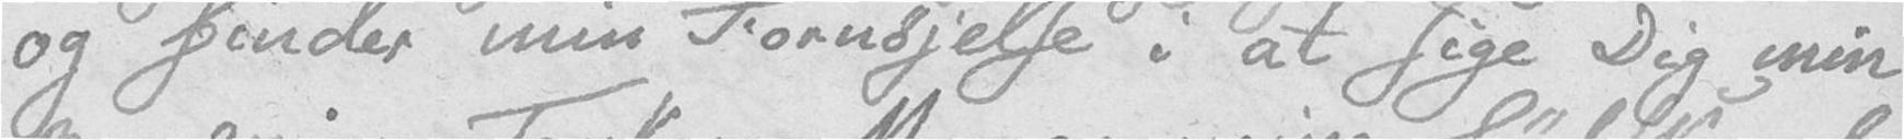og finder min Fornøjelse i at sige Dig min
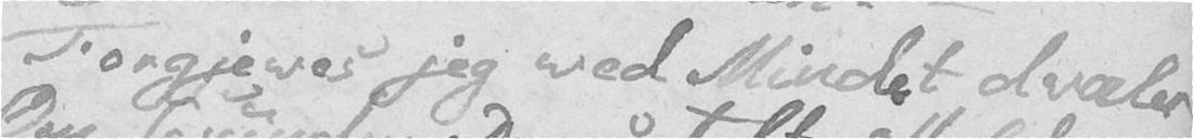Forgjeves jeg ved Mindet dvæler
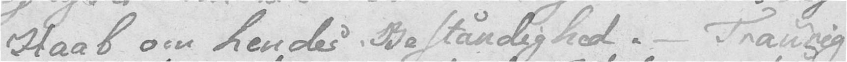Haab om hendes Bestandighed. – Traurig,
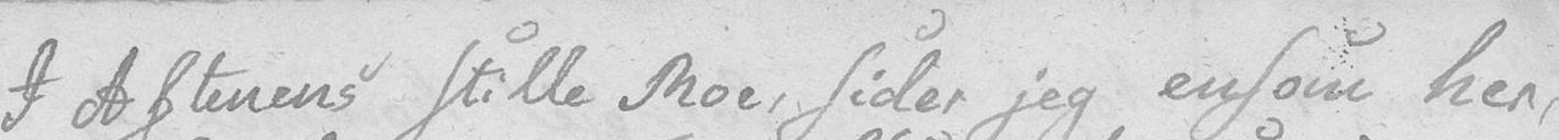I Aftenens stille Roe, sider jeg ensom her,
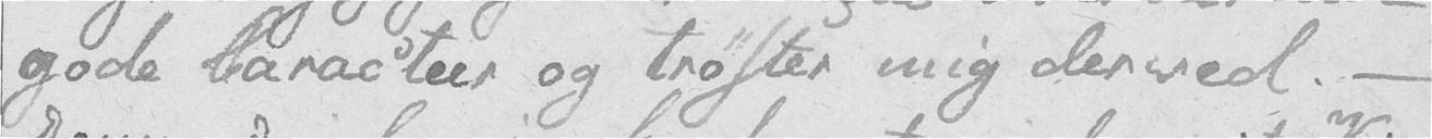gode Caracteer og trøster mig derved. –
